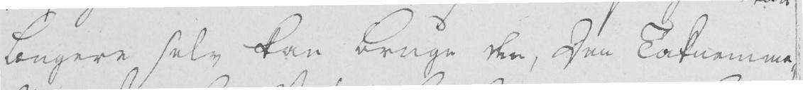længere selv kan bruge den, Den Taknemme-
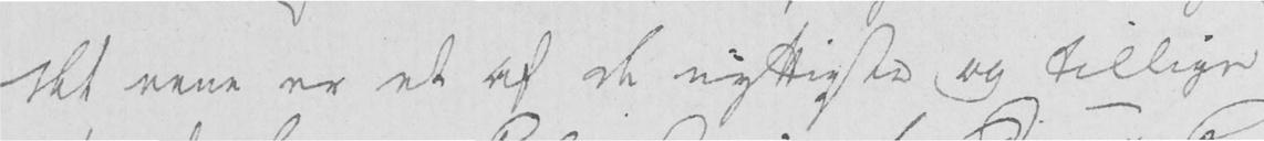det eene er et af de nyttigste og tillige
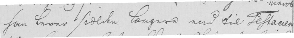han lever siælden længere end til Testamen-
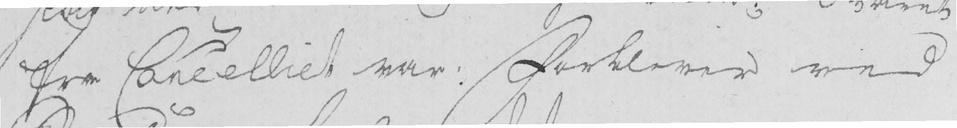fra Cancelliet var: Forbliver ved
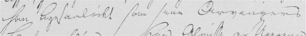han ligesaalidt som sine Arvingers
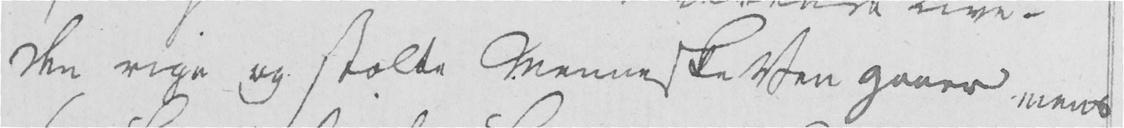den rige og stolte Menneske Ven gaaer mens
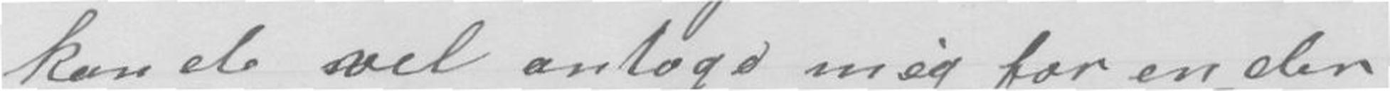kunde vel antage mig for en der
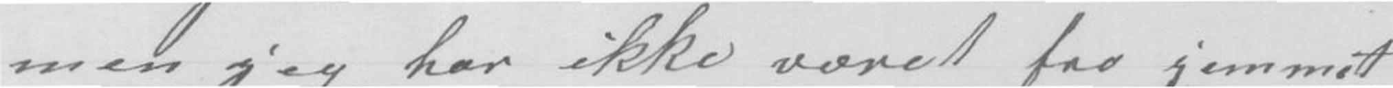men jeg har ikke været fra jemmet
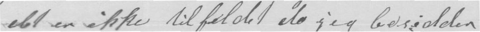det er ikke tilfeldet da jeg besidder
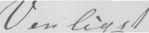Venligst
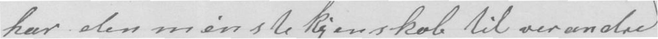har den minste kjenskab til verandre
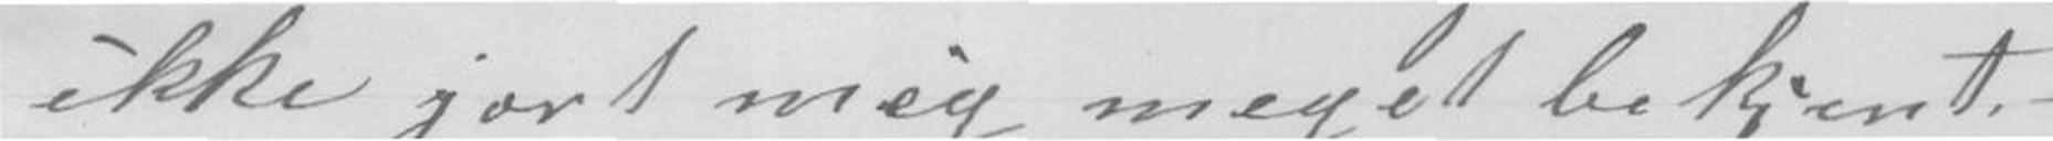ikke jort mig meget bekjent.
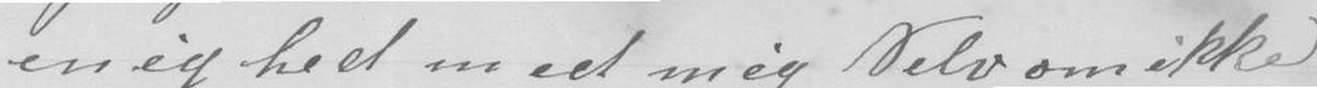enighed med mig Selv om ikke
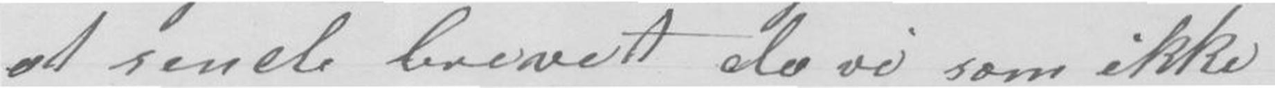at sende brevet da vi som ikke
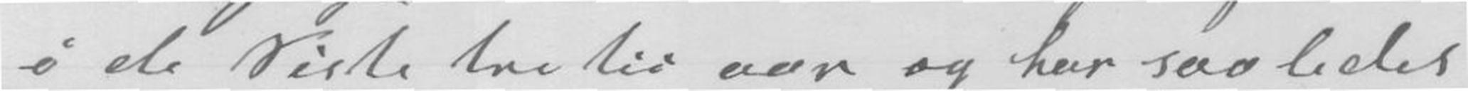i de Siste tretii aar og har saaledes
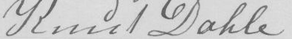Knut Dahle
